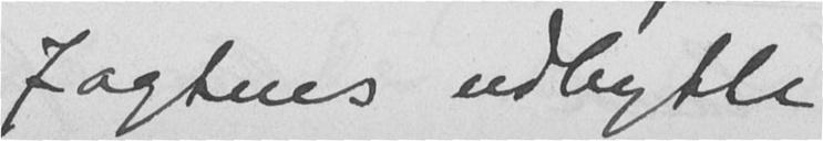Jagtens udbytte
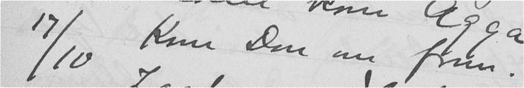17/10 Kom Don om form.
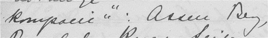kompani": Assen Berg,
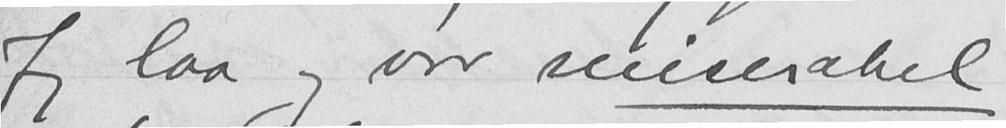Jeg laa og var miserabel
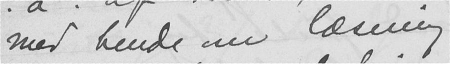med hende om læsning
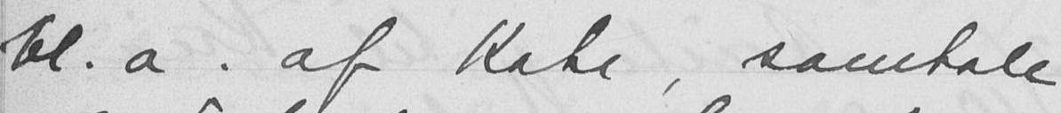bl. a. af Kate, samtale
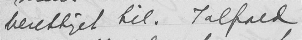berettiget til. Ialfald
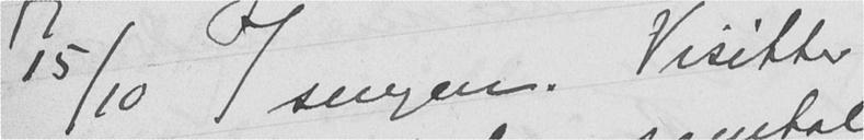15/10 I sengen. Visitter
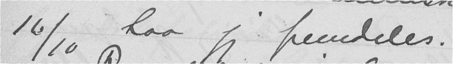16/10 Laa jeg fremdeles.
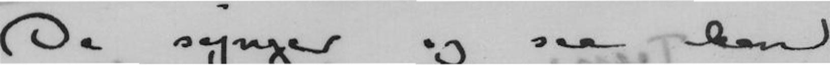De synger og saa kan
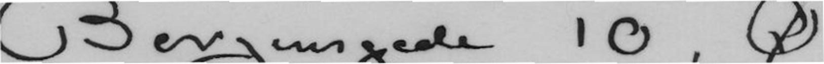Bergensgade 10 , Ø.
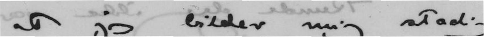at jeg bilder mig stadig
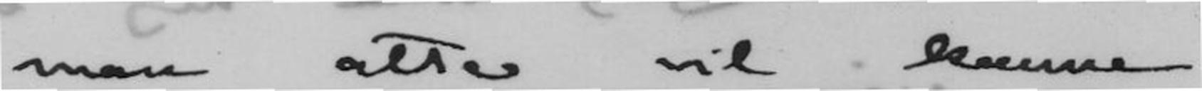man atter vil kunne
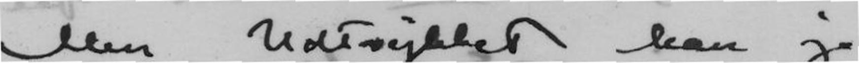Men Udtrykket kan jo
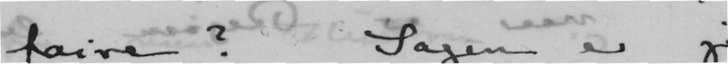faire ? Sagen er jo
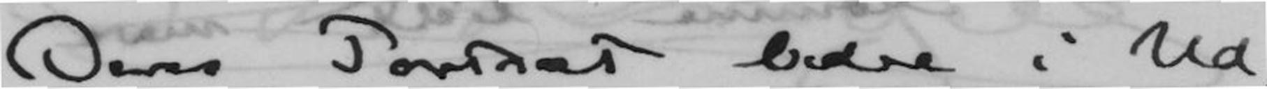Deres Portræt bedre i Ud-
